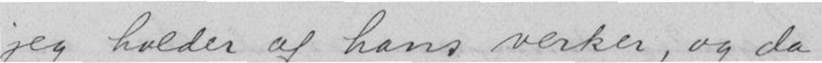jeg holder af hans verker, og da
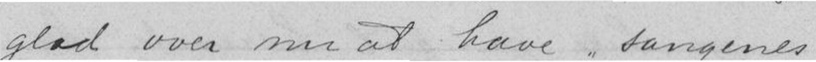glad over nu at have "sangenes
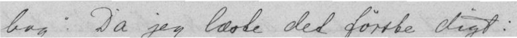bog". Da jeg læste det første digt:
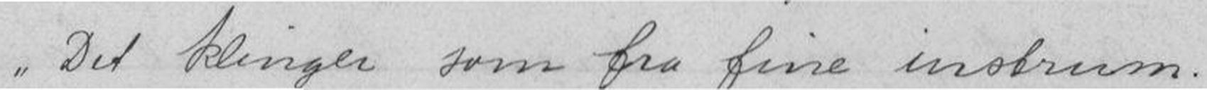"Det klinger som fra fine instrum."
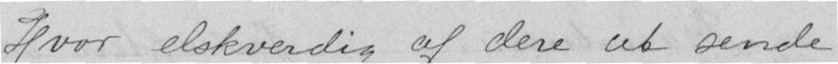Hvor elskverdig af dere at sende
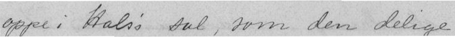oppe i Hals's sal, som den delige
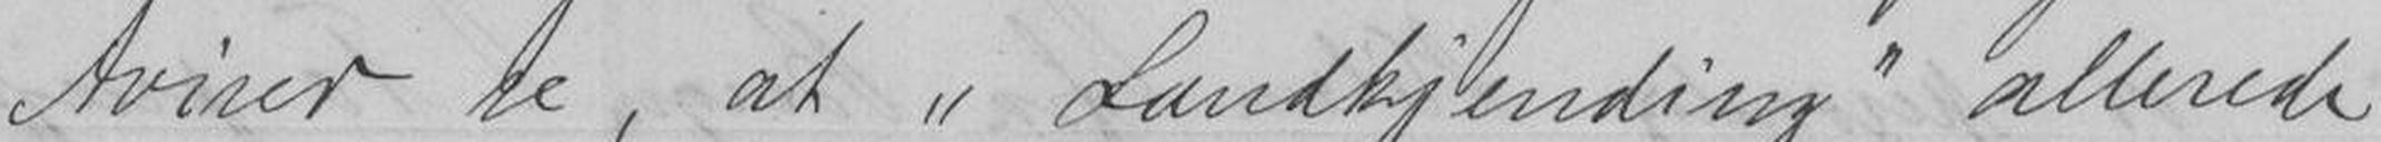Aviser se, at "Landkjending" allerede
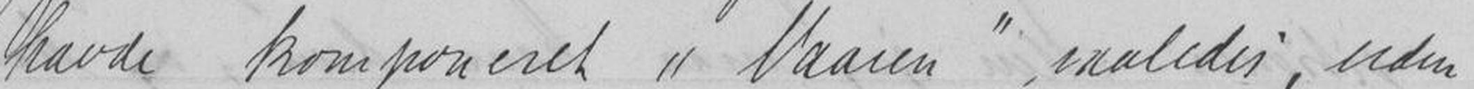havde komponeret "Vaaren" saaledes, uden
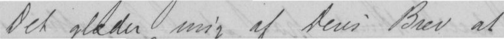Det glæder mig af Deres Brev at
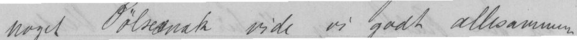noget Pølsesnak vide vi godt allesammen
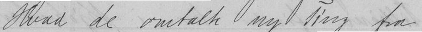Hvad de omtalte ny Ting fra
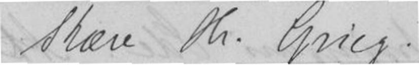Kære Hr. Grieg!
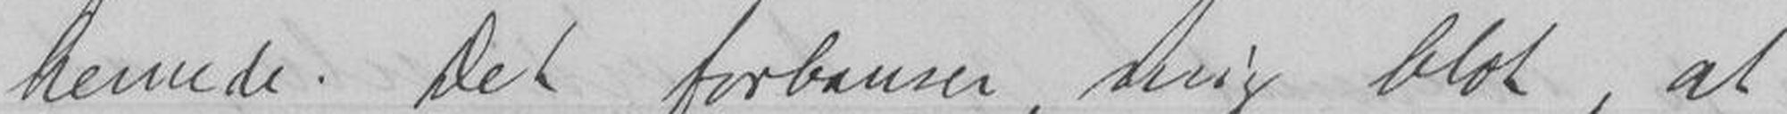henede. Det forbanner mig blot, at
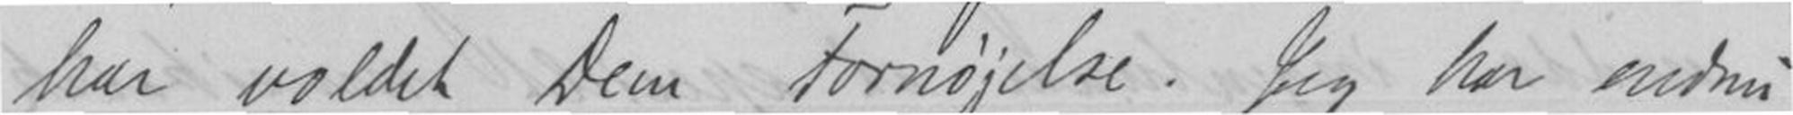har voldet Dem Fornøjelse. Jeg har endnu
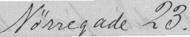Nörregade 23
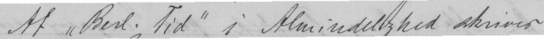At "Berl. Tid" i Almindelighed skriver
